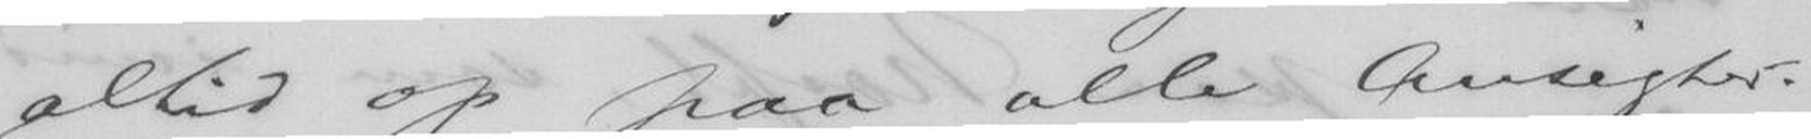altid op paa alle Ansigter.
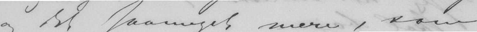og det saameget mere, som
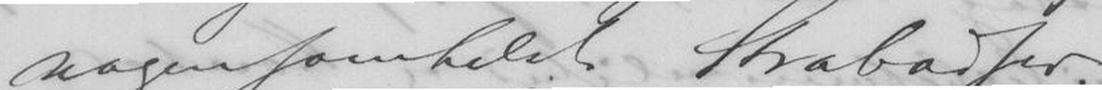nogensomhelst Strabadser. -
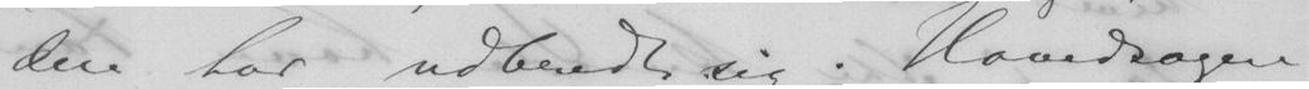den har udbredt sig. Hovedsagen
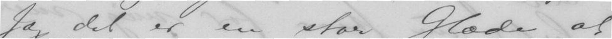Jag det er en stor Glæde at
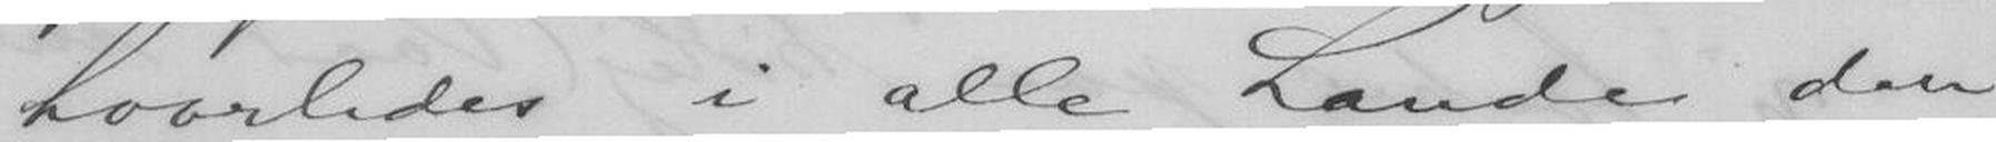hvorledes i alle Lande den
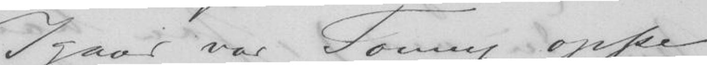Igaar var Tonny oppe
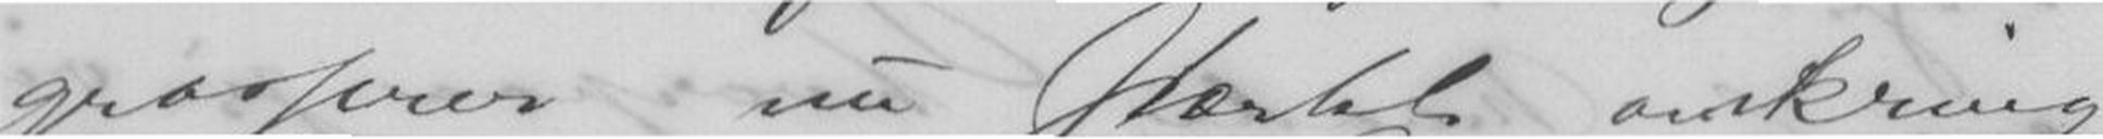grasserer nu stærkt omkring
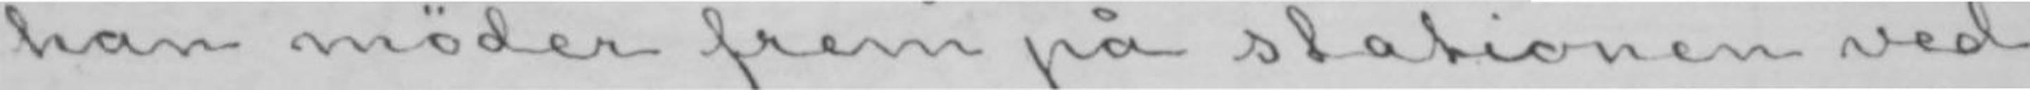han møder frem på stationen ved
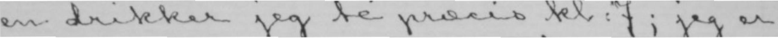en drikker jeg té præcis kl: 7; jeg er
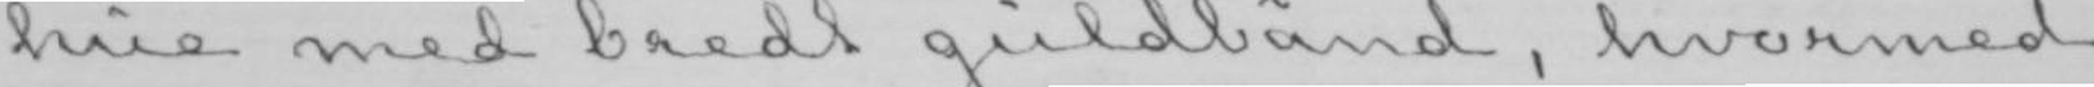lue med bredt guldbånd, hvormed
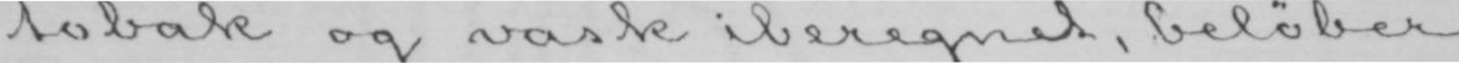tobak og vask iberegnet, beløber
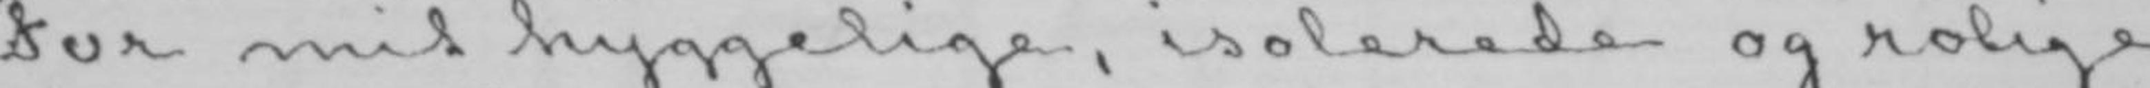For mit hyggelige, isolerede og rolige
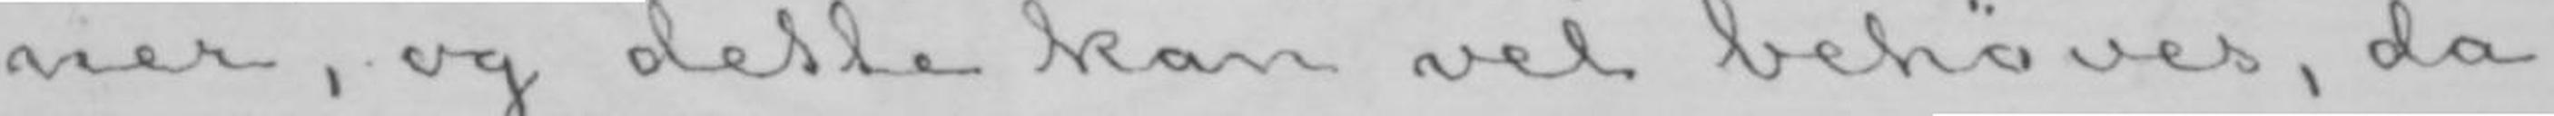ner, og dette kan vel behøves, da
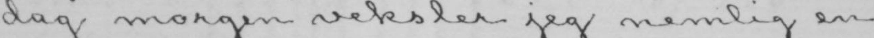dag morgen veksler jeg nemlig en
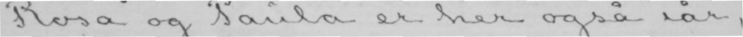- Rosa og Paula er her også iår,
